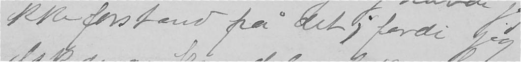ikke forstand på det; fordi jeg
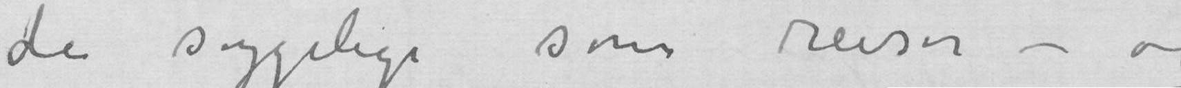de sygelige som reiser – og
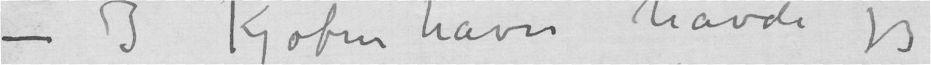– I Kjøbenhavn havde jeg
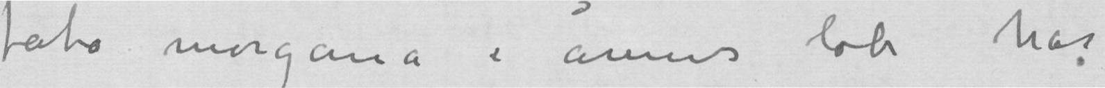fata morgana i årenes løb har
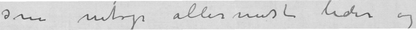som netop aller mest lider og
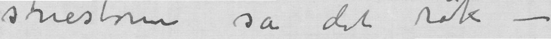snestorm så det røk –
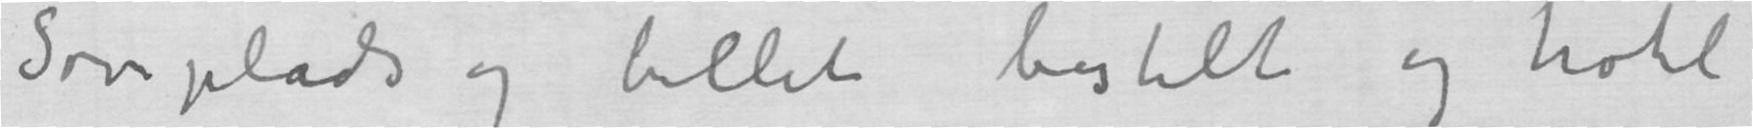Soveplads og billet bestilt og hotel
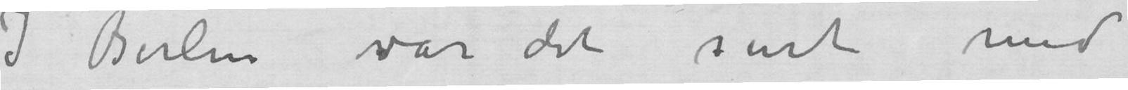I Berlin var det surt med
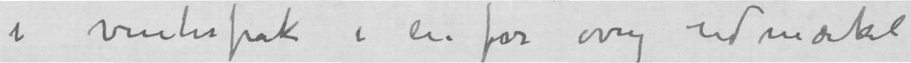i vinterfrak i en for øvrig udmærket
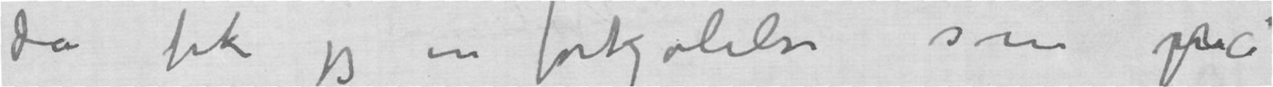da fik jeg en forkjølelse som på
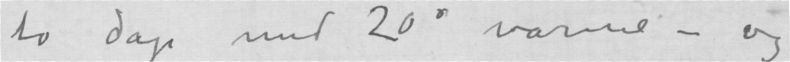to dage med 20° varme – og
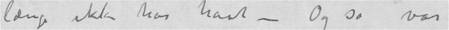længe ikke har havt – Og så var
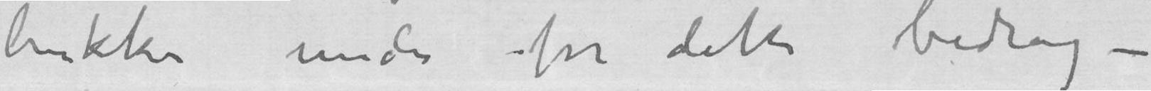bukker under for dette bedrag –
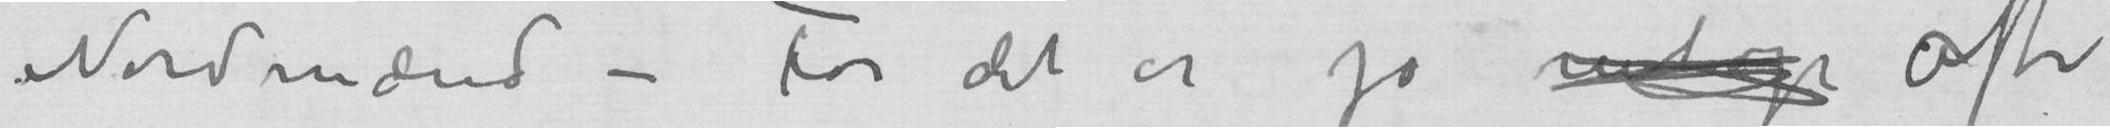Nordmænd – For det er jo netop ofte
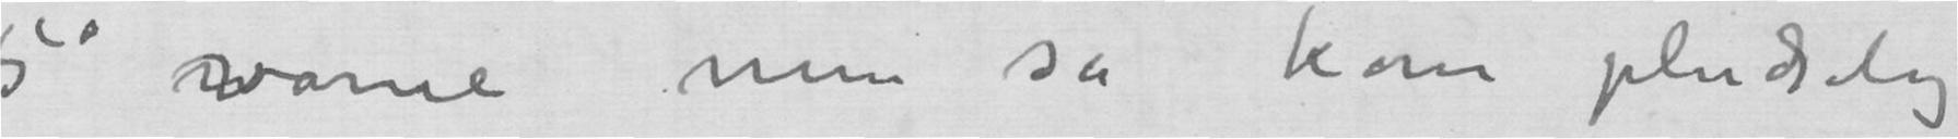5° varme men så kom pludselig
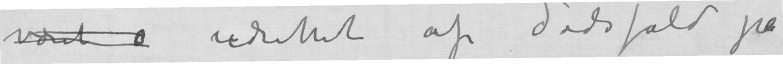værtudrettet af dødsfald på
